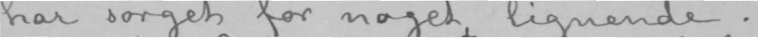har sørget for noget lignende.
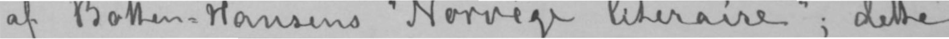af Botten-Hansens «Norvège literaire»; dette
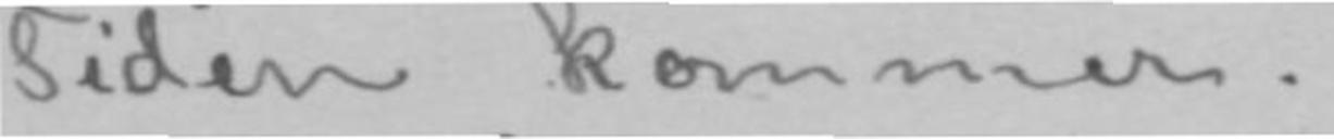Tiden kommer.
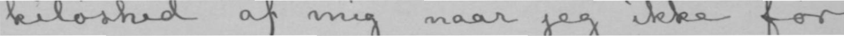keløshed af mig naar jeg ikke før
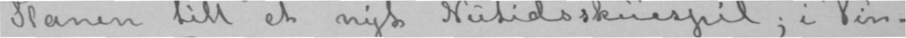Planen till et nyt Nutidsskuespil; i Vin-
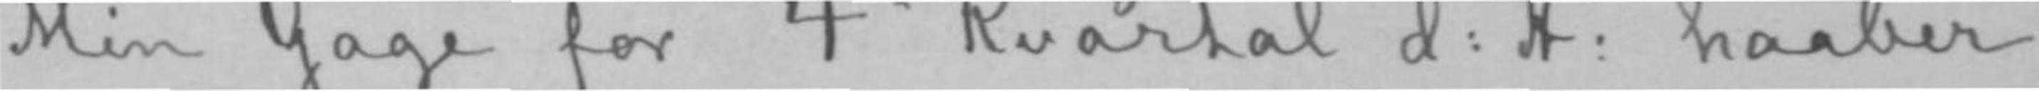Min Gage for 4de Kvartal d: A: haaber
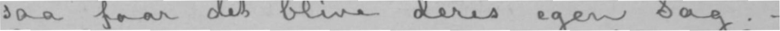saa faar det blive deres egen Sag.-
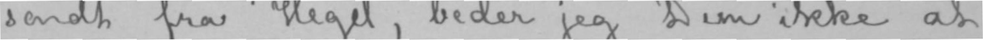sendt fra Hegel, beder jeg Dem ikke at
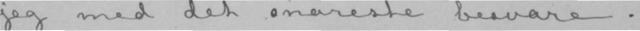jeg med det snareste besvare.
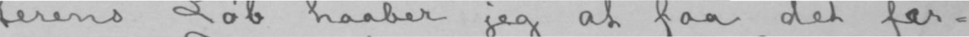terens Løb haaber jeg at faa det fær-
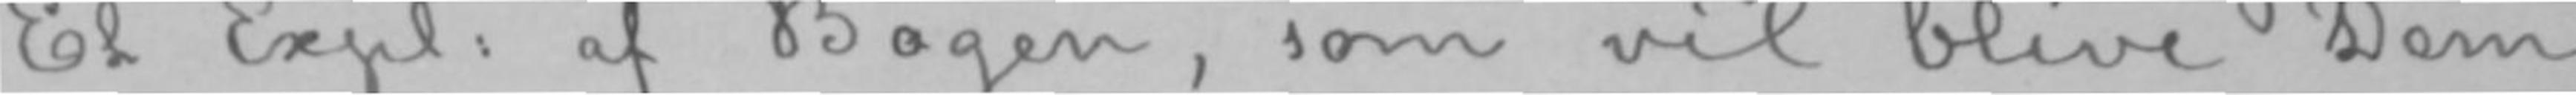Et Expl: af Bogen, som vil blive Dem
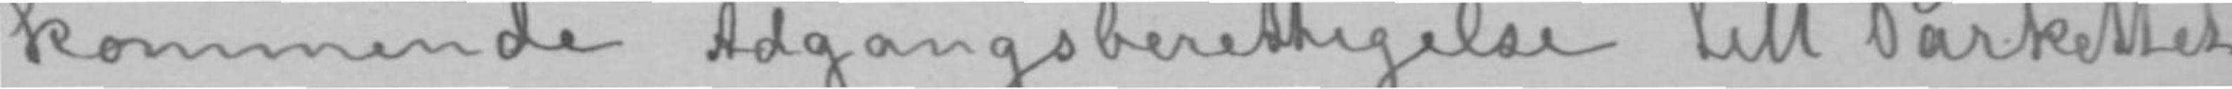kommende Adgangsberettigelse till Parkettet
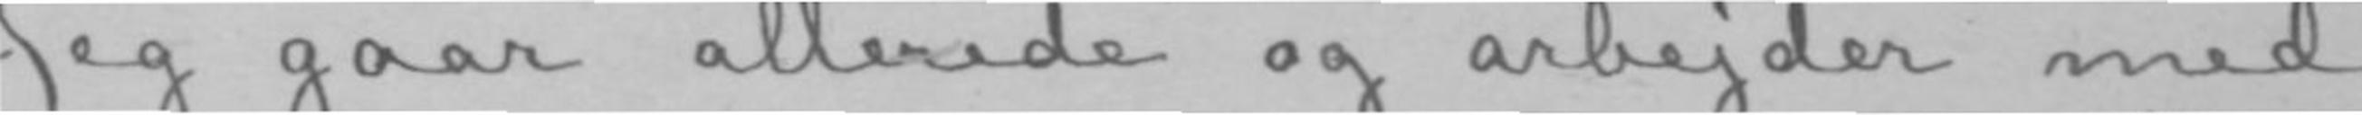Jeg gaar allerede og arbejder med
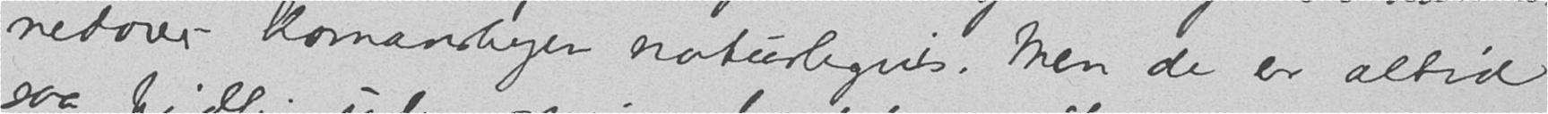nedover Homansbyen naturligvis. Men de er altid
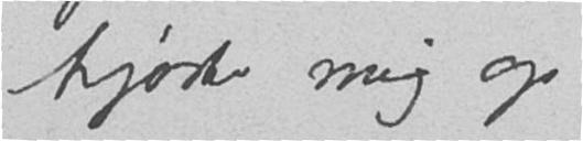kjørte mig op
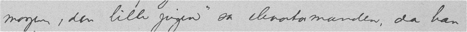morgen, den lille pigen" sa elevatormanden, da han
